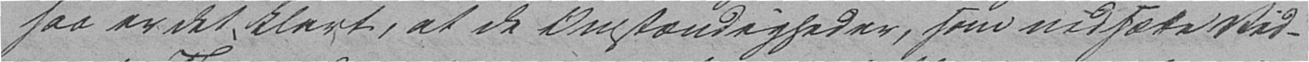saa er det klart, at de Omstændigheder, som nedsætte Vid-
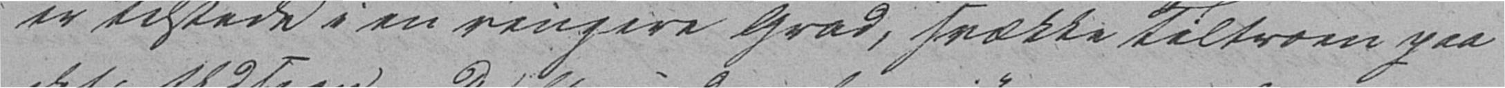er tilstede i en ringere Grad, svække tiltroen paa
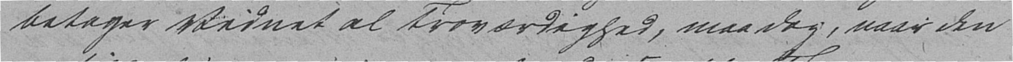betager Vidnet al Troværdighed, maa dog, naar den
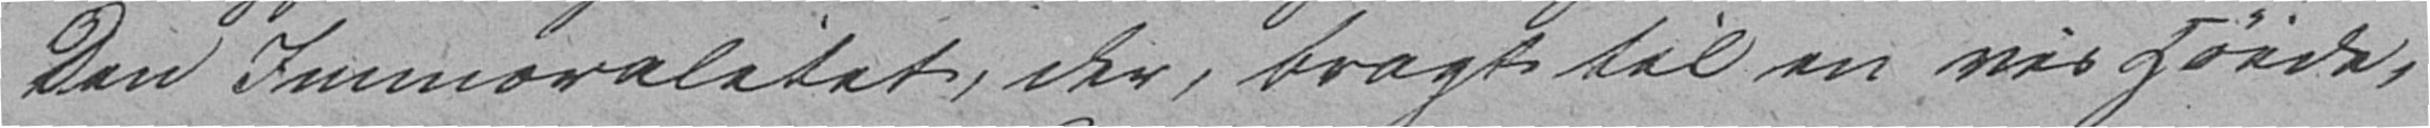Den Immoralitet, der, bragt til en vis Høide,
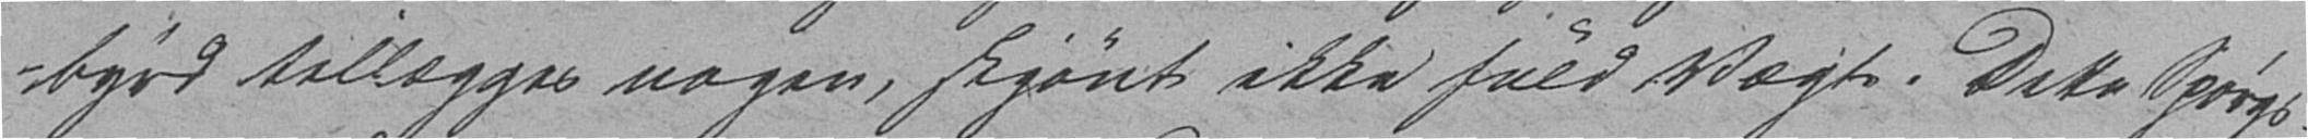byrd tillægges nogen, skjønt ikke fuld Vægt. Dette Spørgs-
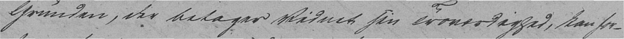Grunden, der betager Vidnet sin Troværdighed, kan for-
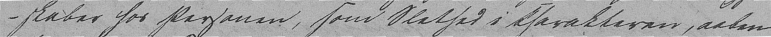skaber hos Personen, som Slethed i Charakteren, aaben-
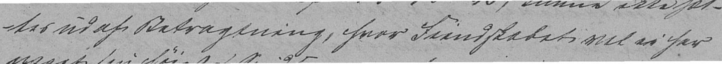tes ud af Betragtning, hvor Fiendskabet vel ei har
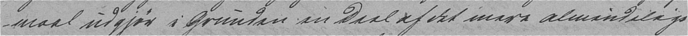maal udgjør i Grunden en Deel af det mere almindelige
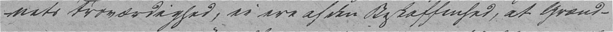nets Troværdighed, ei ere af den Beskaffenhed, at Grænd-
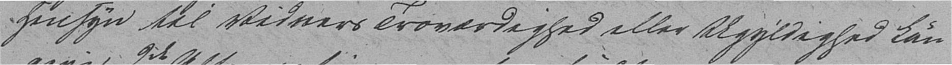Hensyn til Vidners Troværdighed eller Ugyldighed kan
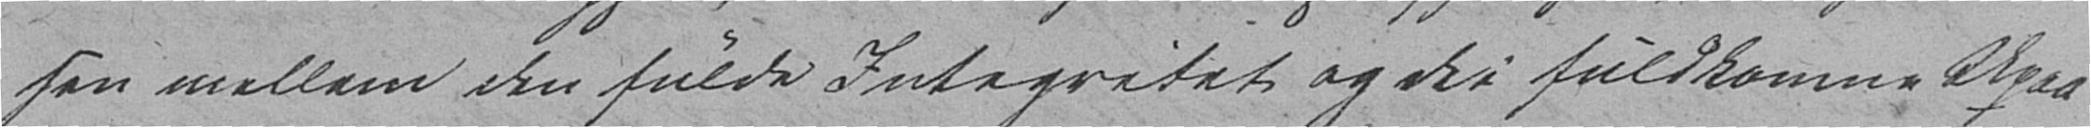sen mellem den fulde Integritet og det fuldkomne Upaa-
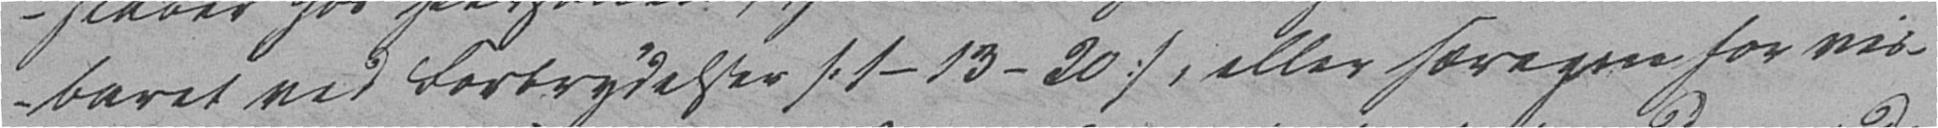baret ved Forbrydelser /: 1-13-20 :/, eller særegen for vis-
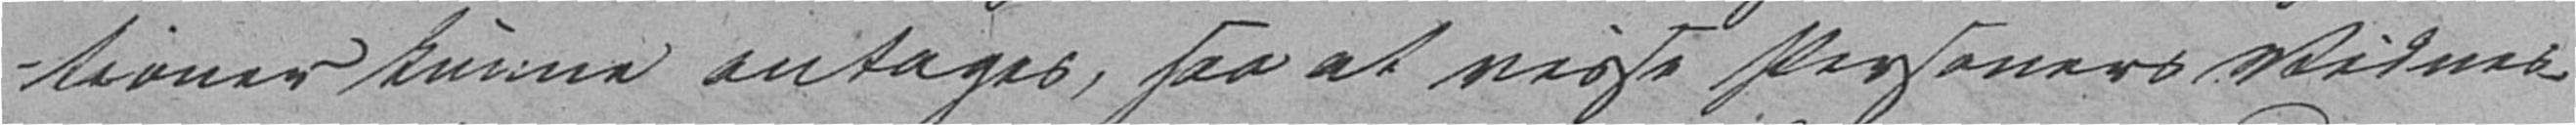tioner kunne antages, saa at visse Personers Vidnes-
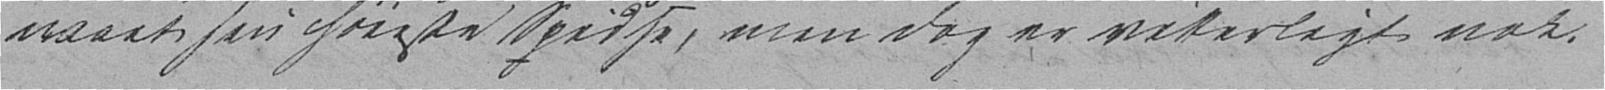naaet sin høieste Spidse, men dog er vitterligt nok.
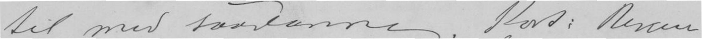til med saadanne. Kort; Bergen
